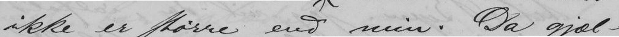ikke er større end min. Da gjæl-
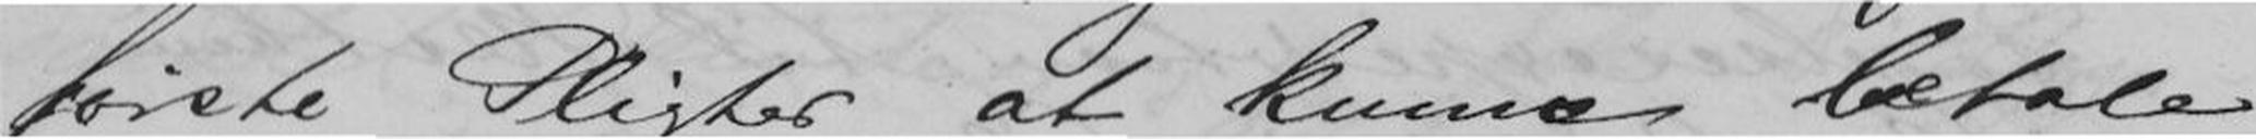første Pligter at kunne betale
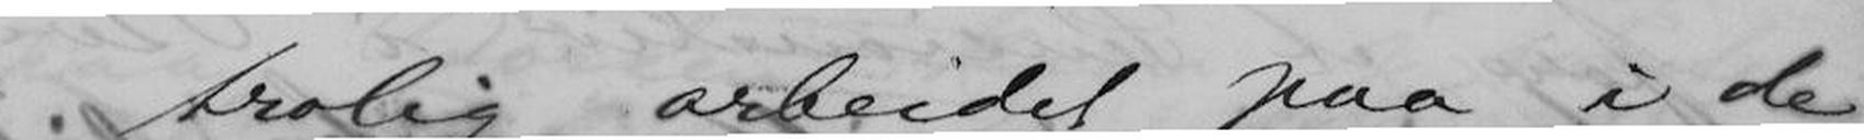og jeg trolig arbeidet paa i de
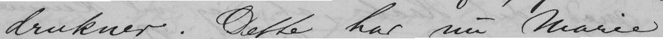drukner. Dette har nu Marie
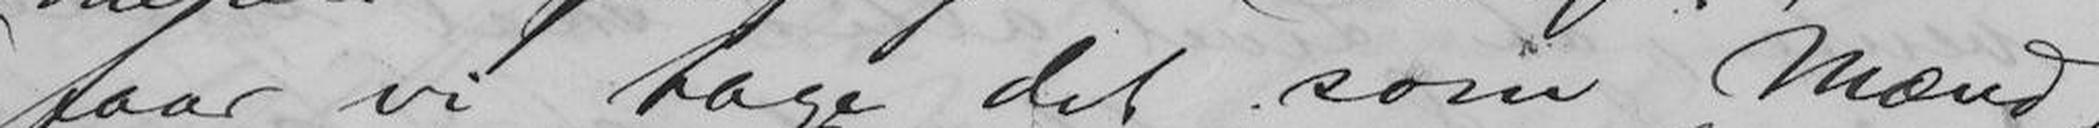faar vi tage det som Mænd,
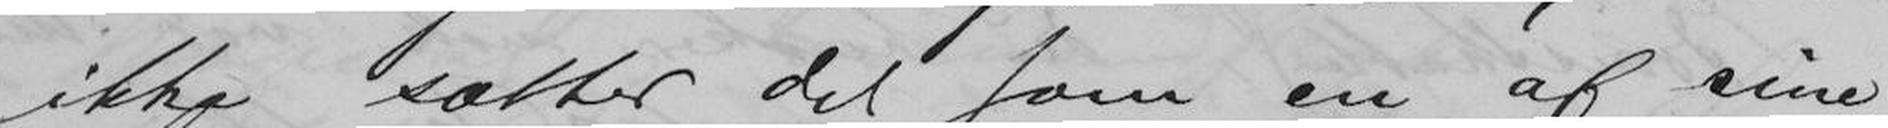ikke sætter det som en af sine
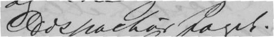Dispachørfaget.
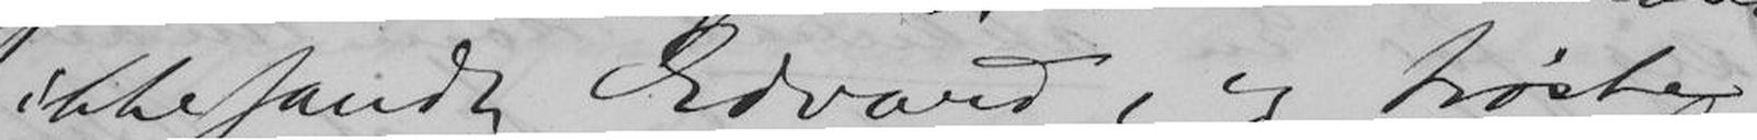ikkesandt Edvard, og trøste
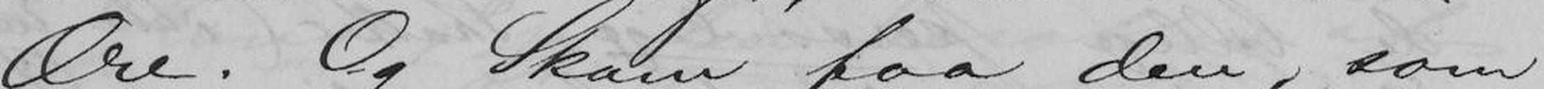Ære. Og Skam faa den, som
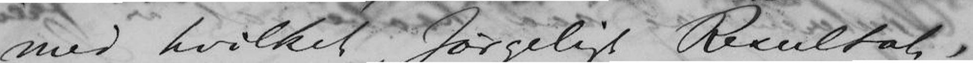med hvilket sørgeligt Resultat,
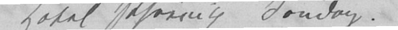Hotel Phøenix Søndag.
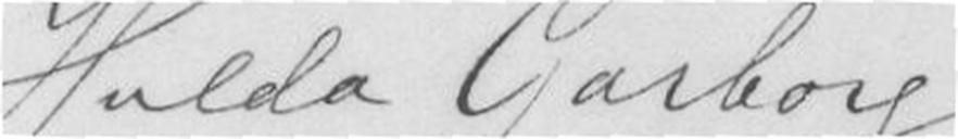Hulda Garborg
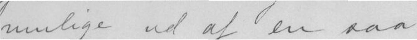mulige ud af en saa
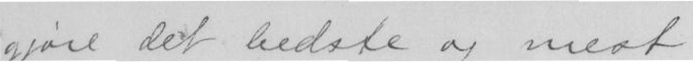gjøre det bedste og mest
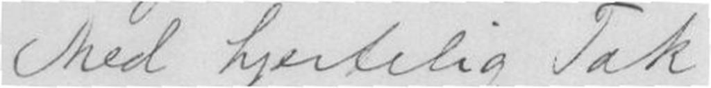Med hjertelig Tak
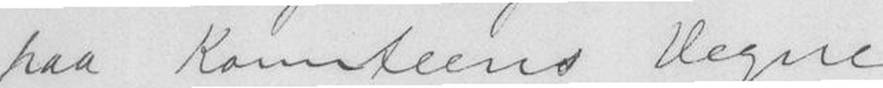paa Komiteens Vegne
